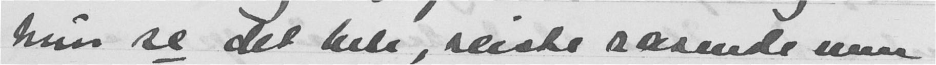hun se det hele, reiste rasende men
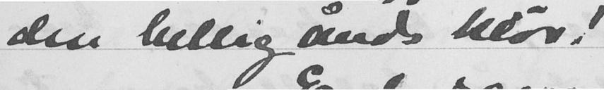den hellig ånds klør!
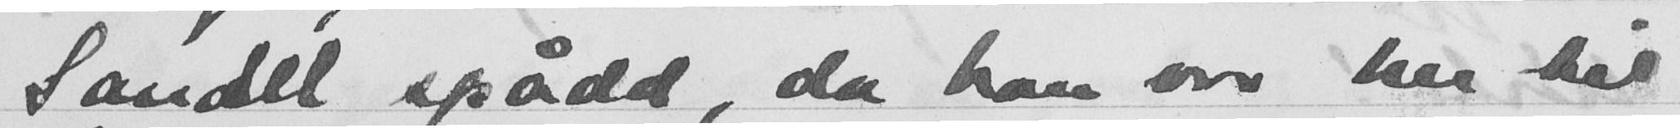Sandel spådd, da han var her til
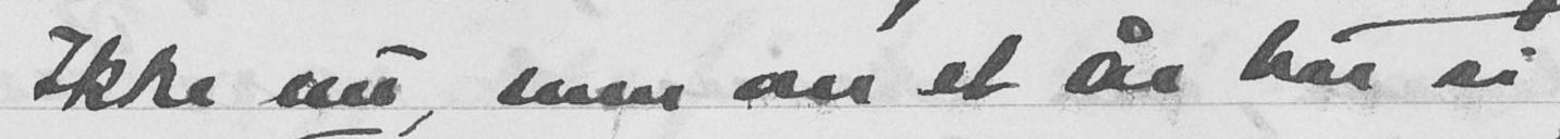Ikke nu, men om et år har vi
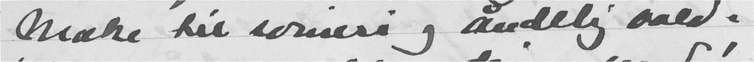Make til svineri og åndelig vold-
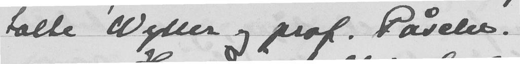talte Wyller og prof. Påsche.
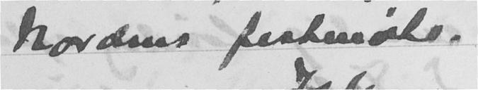Nordens festmøte.
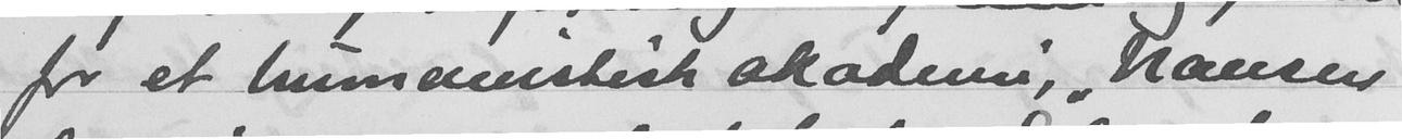for et humanistisk akademi, "Nansen
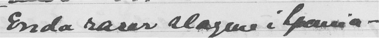Enda raser slagene i Spania -
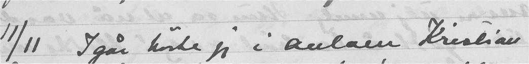11/11 Igår hørte jeg i aulaen Kristian
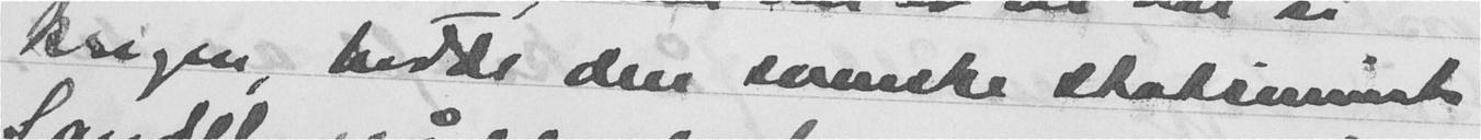krigen, hadde den svenske statsminister
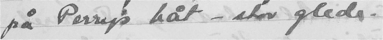på Perrys båt - stor glede.
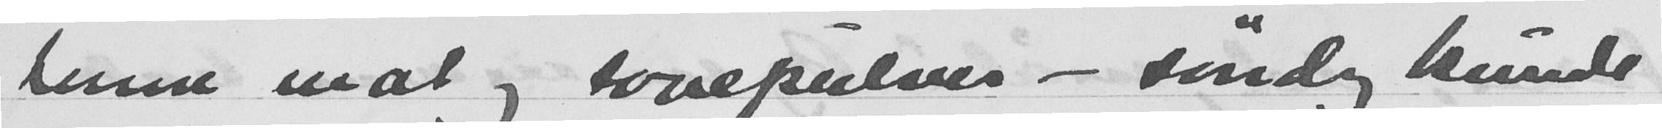henne mat og løvepulver - søndag kunde
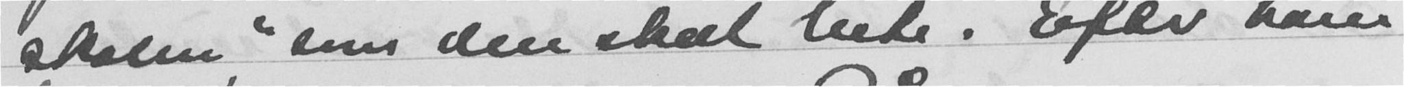skolen" som den skal hete. Efter ham
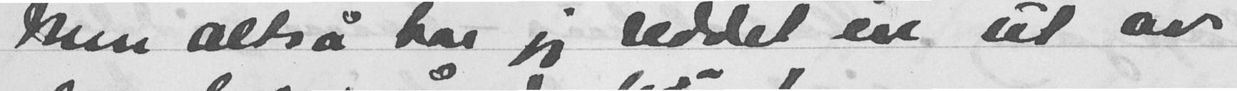Men altså har jeg reddet én ut av
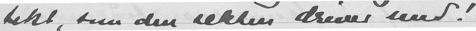tekt, som den sekten driver med!
